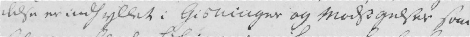delse er indhyllet i Gisninger og Modsigelser som
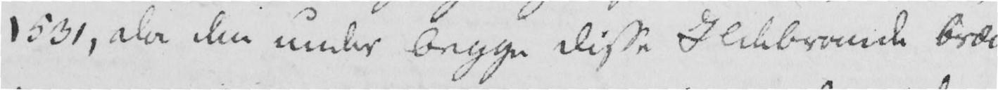1531, da den under begge disse Ildebrande bræn-
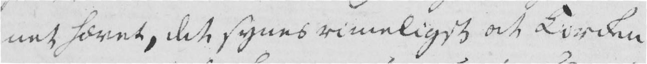net hævet, det synes rimeligst at Kirken
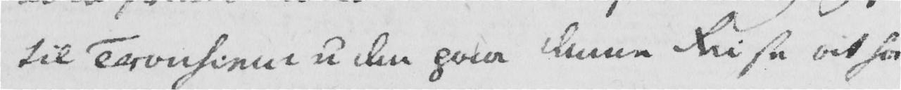til Tronhiem uden paa denne Reise at ha-
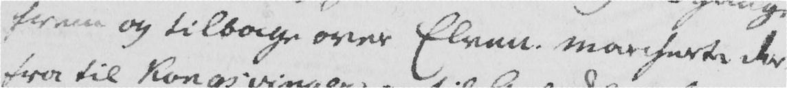frem og tilbage over Elven. marcherte der-
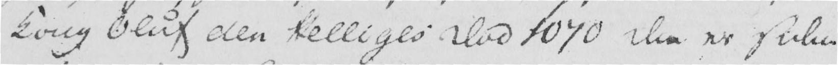Kong Oluf den Helliges død 1070 Den er siden
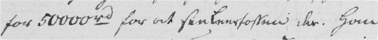for 50000 rd for at see Leerfossen der. Han
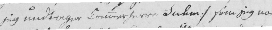jeg undtager Kammerherre Suhm :/ som jeg no-
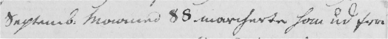Septemb. Maaned 88 marcherte han ud fra
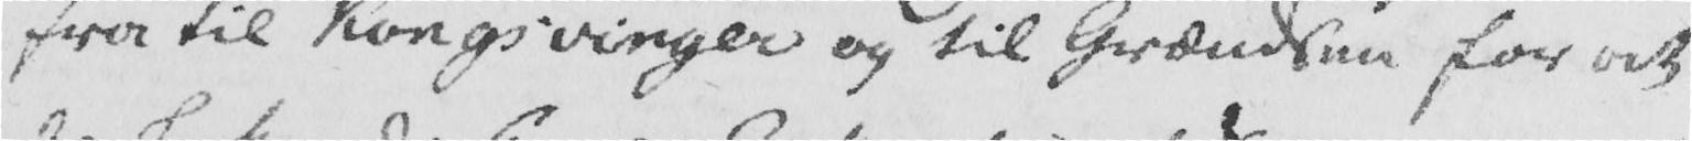fra til Kongsvinger og til Grændsen for at
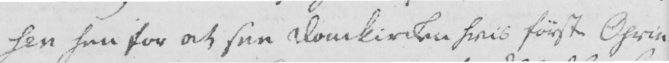sen hen for at see domkirken hvis første Oprin-
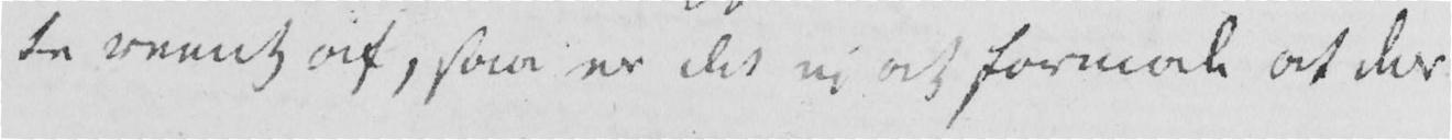te reent af, saa er det ei at formode at der
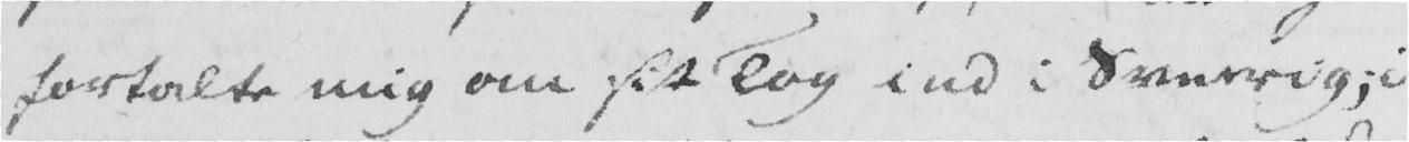fortalte mig om sit Tog ind i Sverrig; i
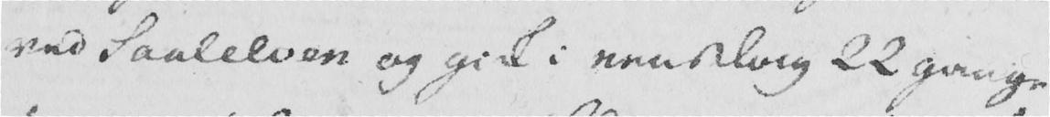ved Saalelven og gik i een Dag 22 gange
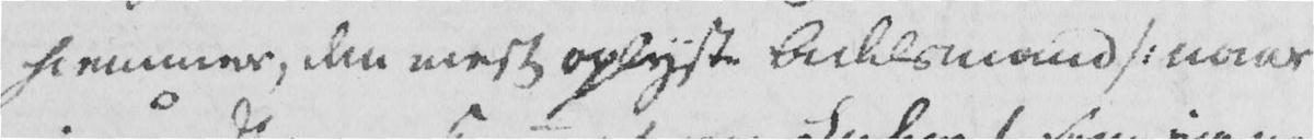hiemmer, den mest oplyste Adelsmand /: naar
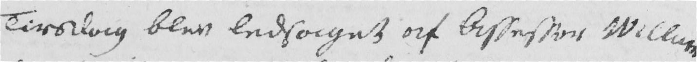Tirsdag blev ledsaget af Assessor Willum-
